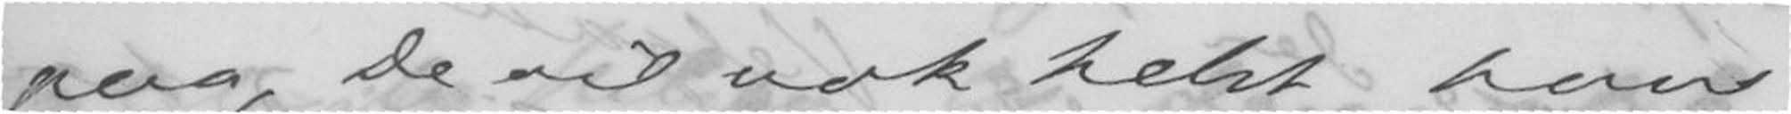paa, De vil nok helst have
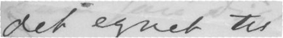det egnet til
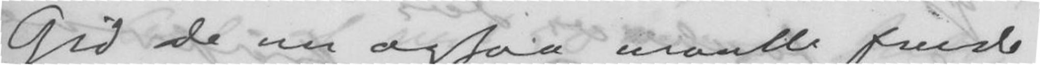Gid de nu ogsaa maatte finde
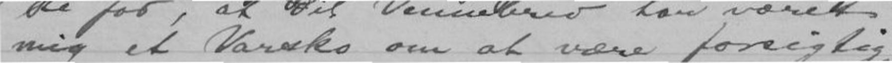mig et Varsko om at være forsigtig
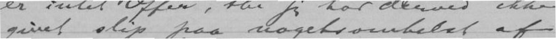givet slip paa nogetsomhelst af
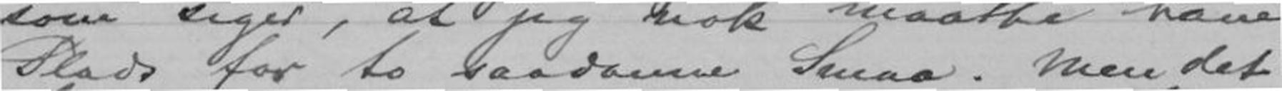Plads for to saadanne Smaa. Men det
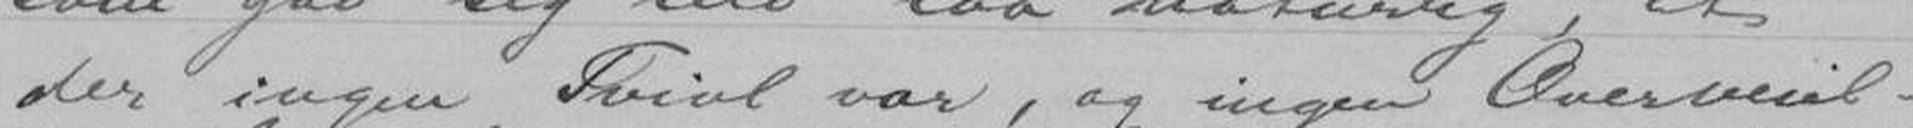der ingen Tvivl var, og ingen Overveiel-
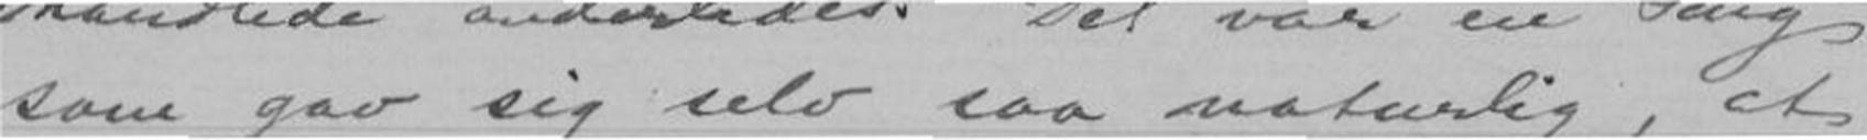som gav sig selv saa naturlig, at
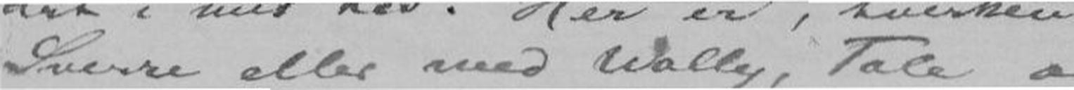Sverre eller med Wally, Tale om
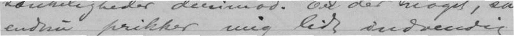endnu prikker mig lidt indvendig,
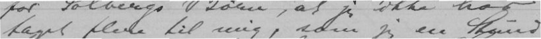taget flere til mig, som jeg en Stund
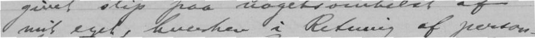mit eget, hverken i Retning af person-
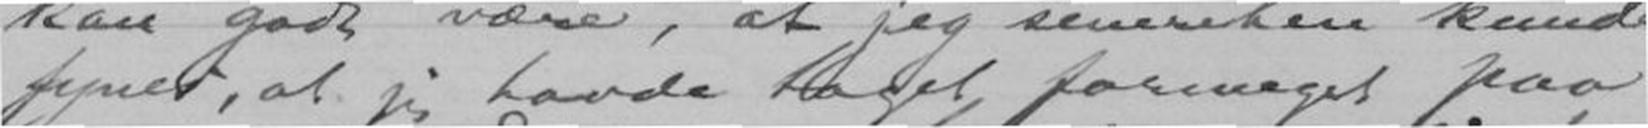synes, at jeg havde taget formeget paa
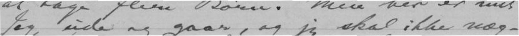Jeg ude og gaar, og jeg skal ikke næg-
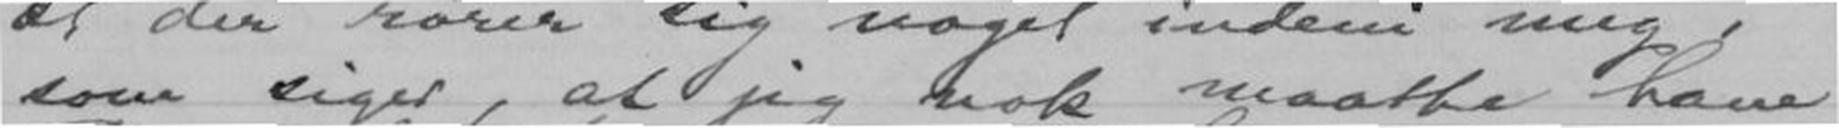som siger, at jeg nok maatte have
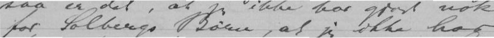for Solbergs Børn, at jeg ikke har
